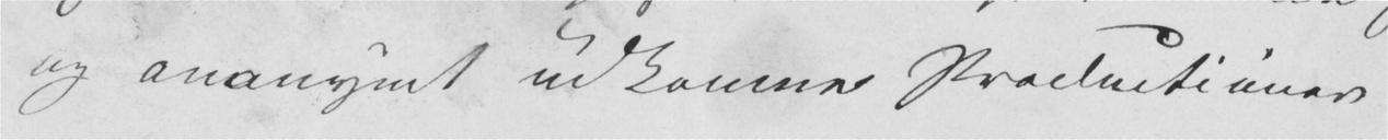og anonymt udkomne Productioner
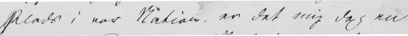Plads i vor Nation, er det mig dog en
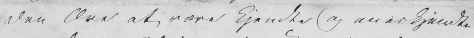den Ære at være kjendte og anerkjendte
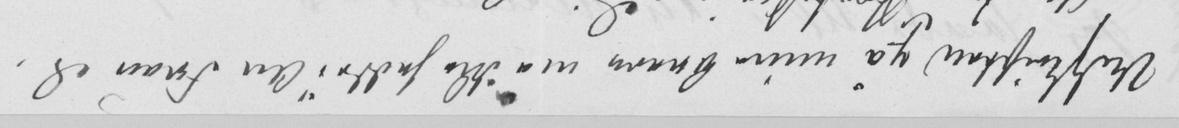Udskriften på mine Breve må ikke hedde: "An Frau etc.
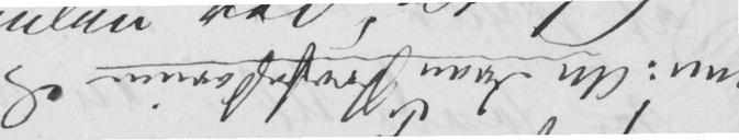men: An Frau Professorinne etc.
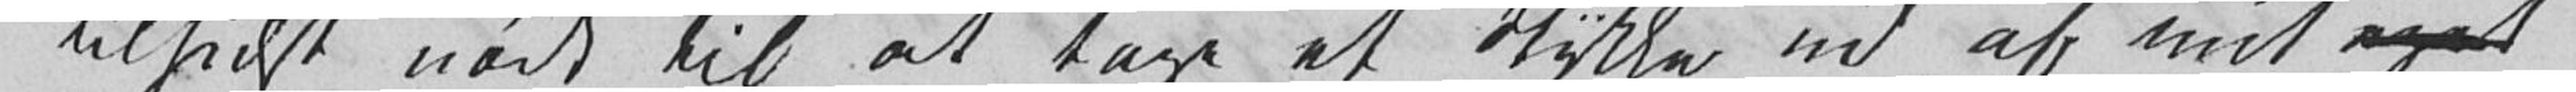tilsidst nødt til at tage et Stykke ud af mit eget
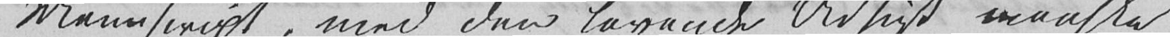Manuscript, med den lovende Udsigt maaske
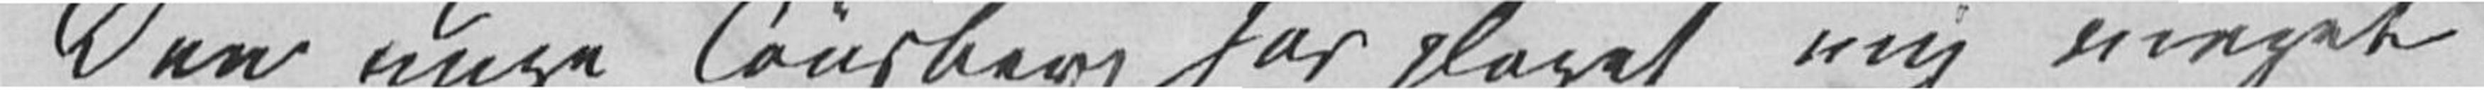Den unge Tønsberg har plaget mig meget
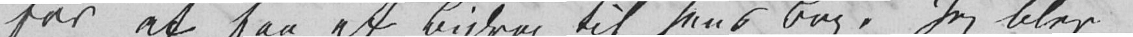for at faa et Bidrag til hans Bog. Jeg blev
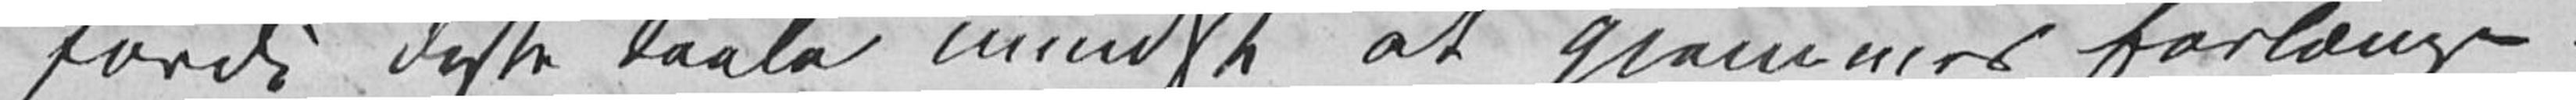fordi disse taale mindst at gjemmes forlænge.
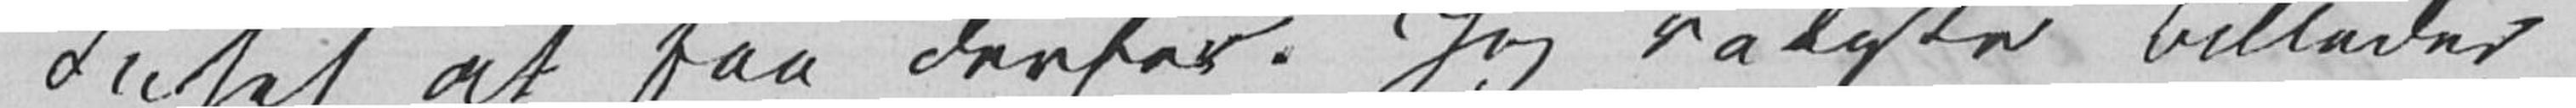Intet at faa derfor. Jeg valgte «Billeder
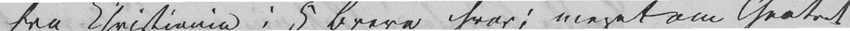fra Christiania i 5 Breve» hvori meget om Theatret
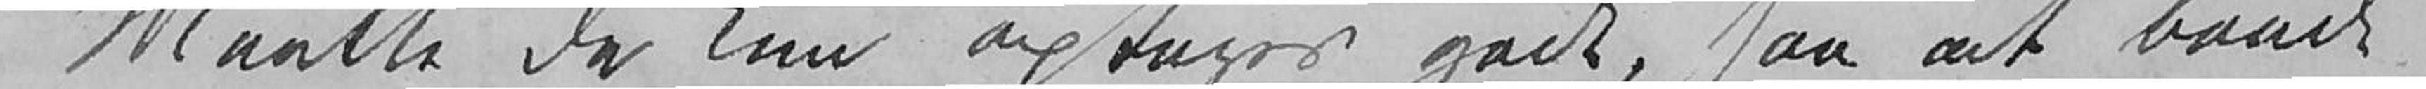Maatte de kun optages godt, saa at baade
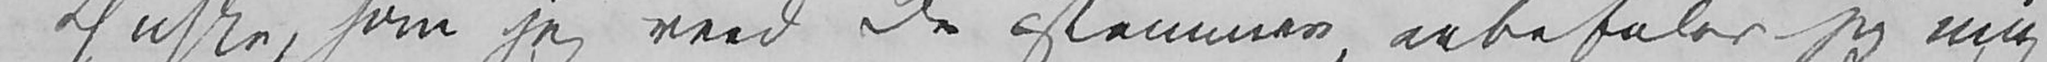Ønske, som jeg veed De istemmer, anbefaler jeg mig
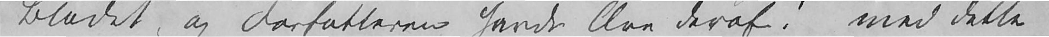Bladet og Forfatteren havde Ære deraf! med dette
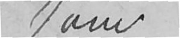som
